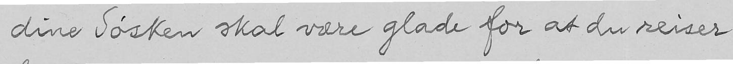dine Søsken skal være glade for at du reiser
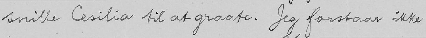snille Cesilia til at graate. Jeg forstaar ikke
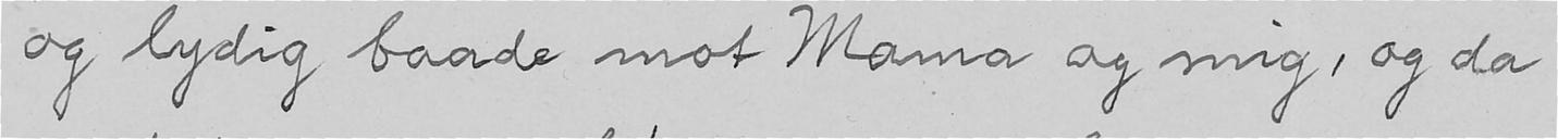og lydig baade mot Mama og mig, og da
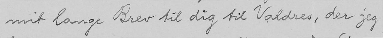mit lange Brev til dig til Valdres, der jeg
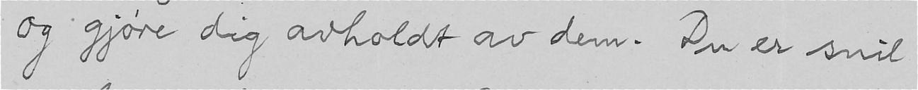og gjøre dig avholdt av dem. Du er snil
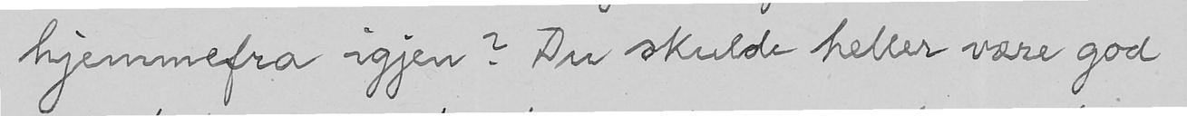hjemmefra igjen? Du skulde heller være god
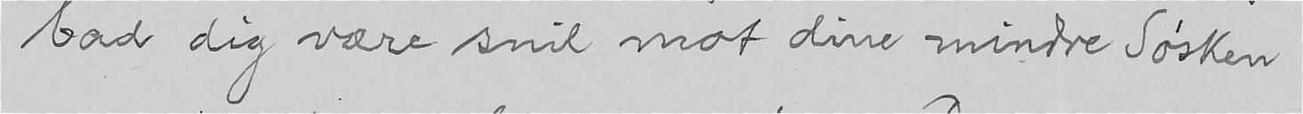bad dig være snil mot dine mindre Søsken
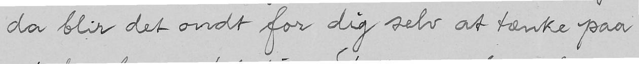da blir det ondt for dig selv at tænke paa
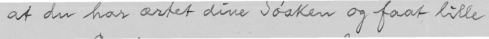at du har ærtet dine Søsken og faat lille
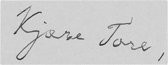Kjære Tore,
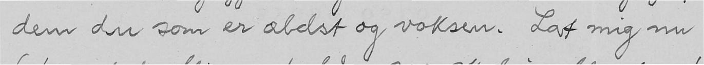dem du som er ældst og voksen. Lat mig nu
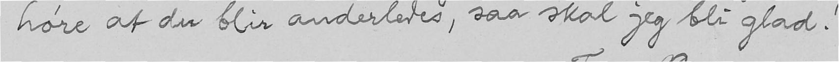høre at du blir anderledes, saa skal jeg bli glad!
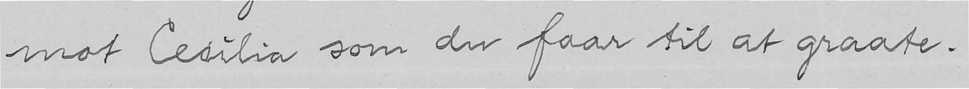mot Cesilia som du faar til at graate.
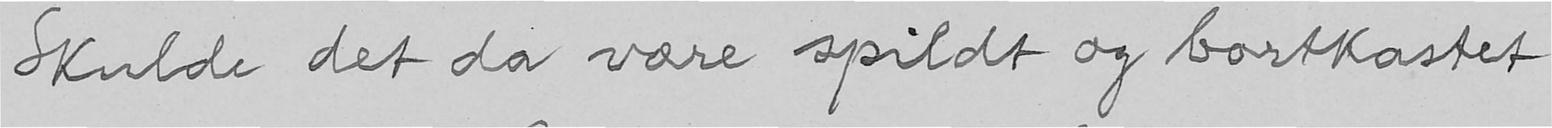Skulde det da være spildt og bortkastet
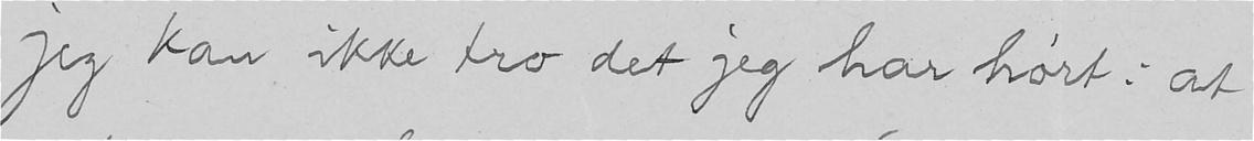jeg kan ikke tro det jeg har hørt: at
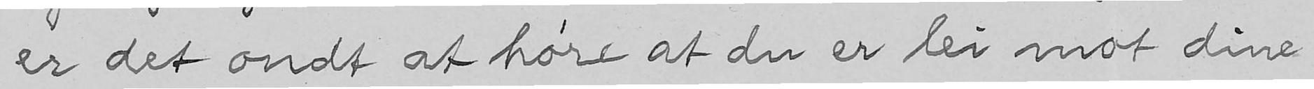er det ondt at høre at du er lei mot dine
